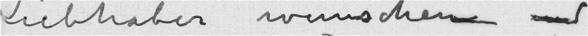Liebhaber wünschen und
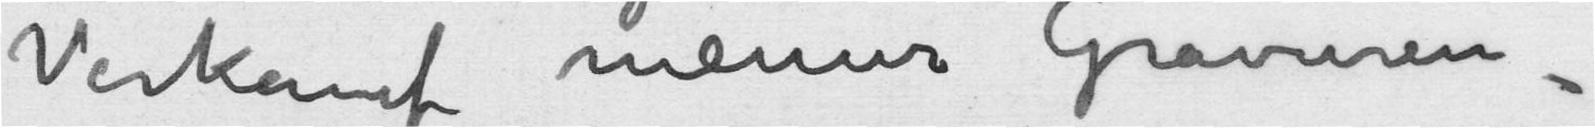Verkauf meiner Gravuren –
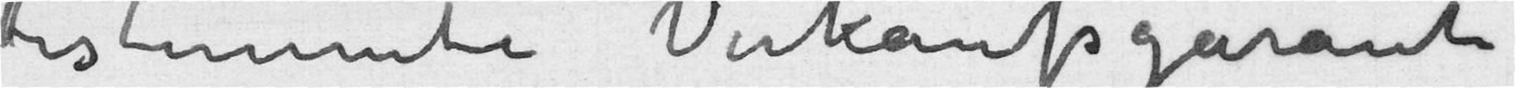bestimmte Verkaufsgaranti
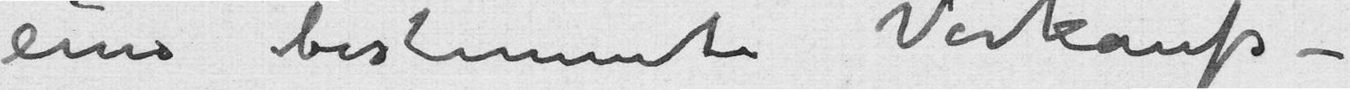eine bestimmte Verkaufs-
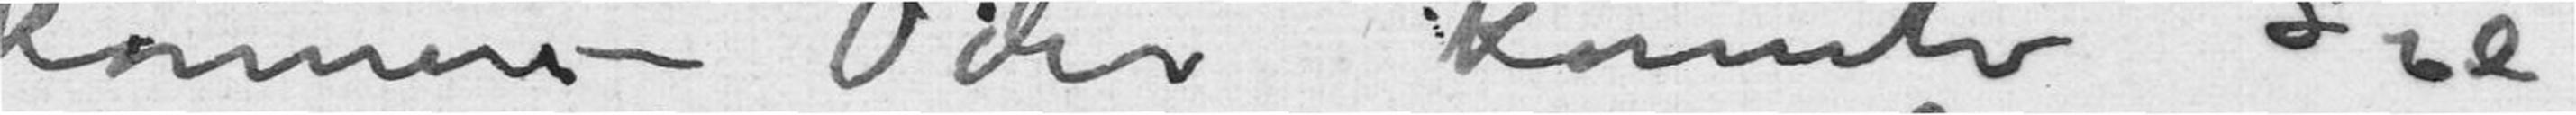können – Oder konnnte Sie
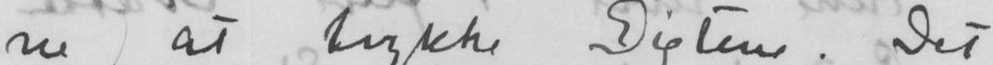ne, at trykke Digtene. Det
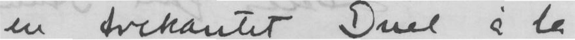en trekantet Duel à la
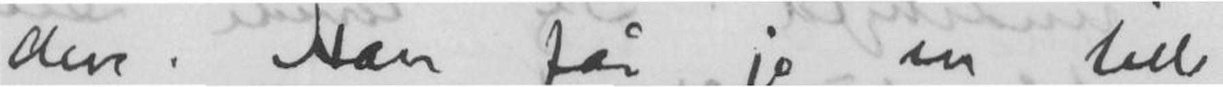dere. Han får jo en lille
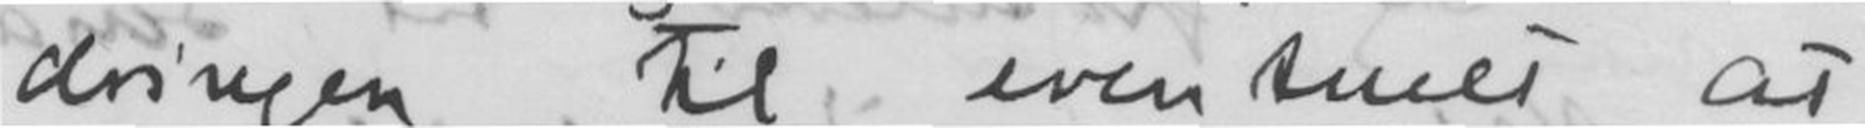dringen til eventuelt at
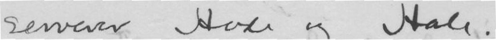serverer Hode og Hale.
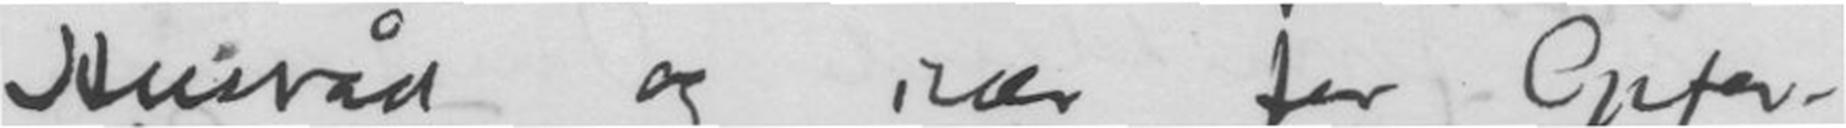Husråd og især for Opfor-
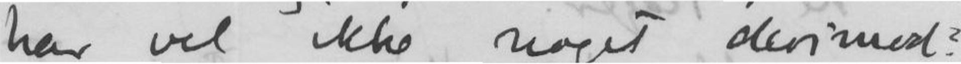har vel ikke noget derimod?
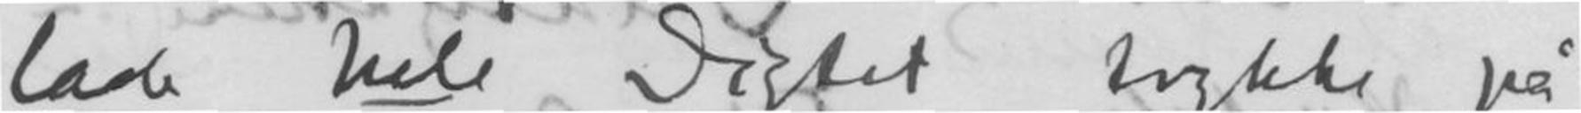lade hele Digtet trykke på
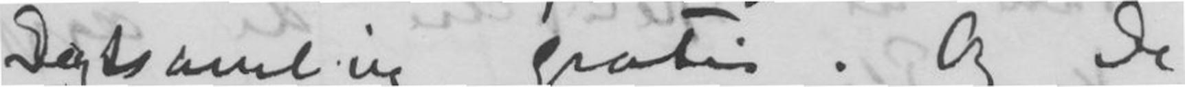Digtsamling gratis. Og De
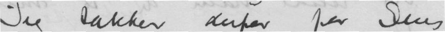Jeg takker derfor for Deres
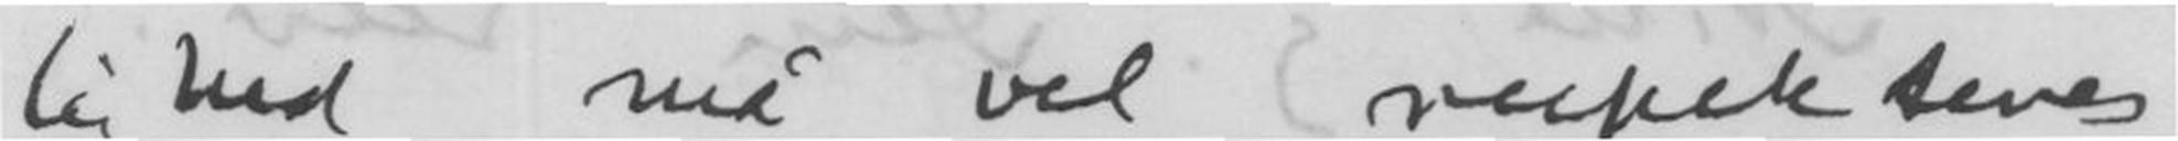lighed må vel respekteres.
